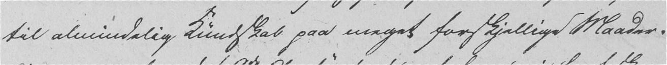til almindelig Kundskab paa meget forskjellige Maader.
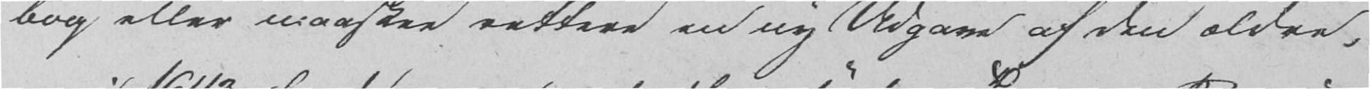bog eller maaskee rettere en ny Udgave af den ældre,
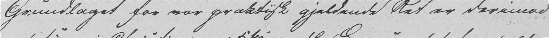Grundlaget for vor praktisk gjeldende Ret er derimod
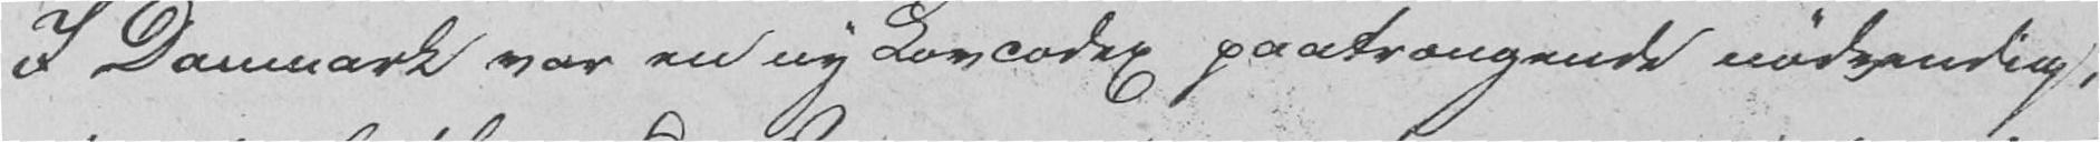I Danmark var en ny Lovcodex paatrængende nødvendig,
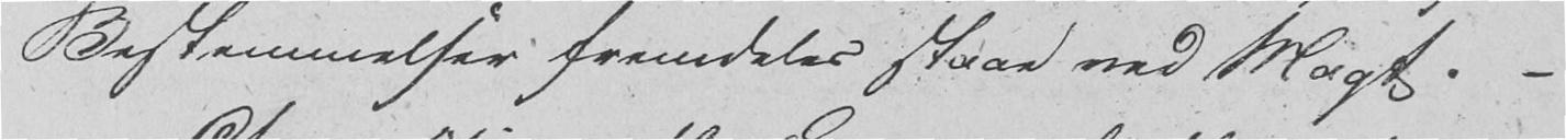Bestemmelser fremdeles staar ved Magt. -
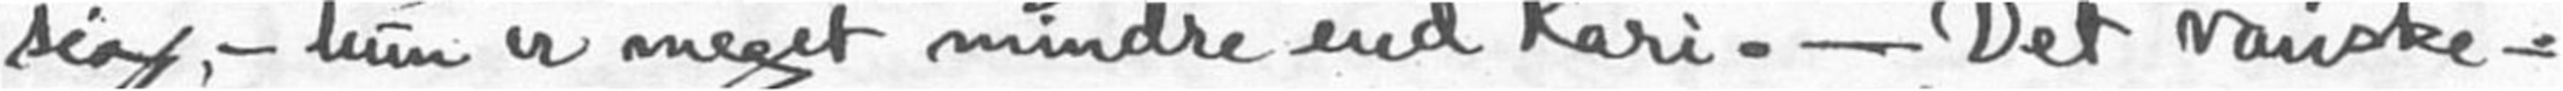sig, - hun er meget mindre end Kari. - Det vanske-
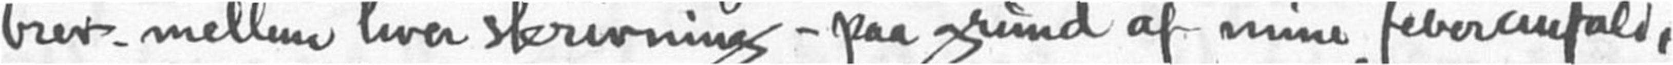brev - mellem hver skrivning - paa grund af mine feberanfald,
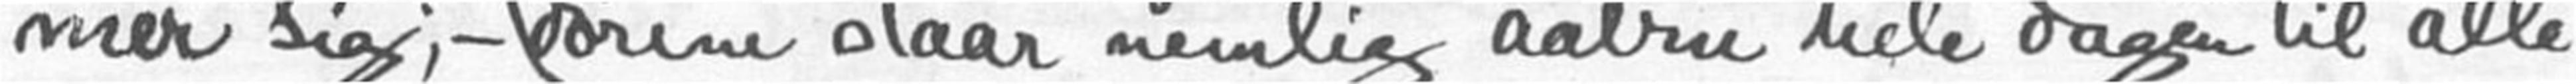mer sig ; - (dørene staar nemlig aabne hele dagen til alle
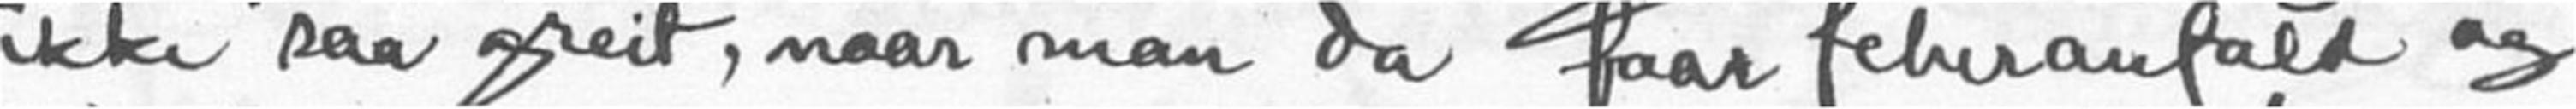ikke saa greit, naar man da faar feberanfald og
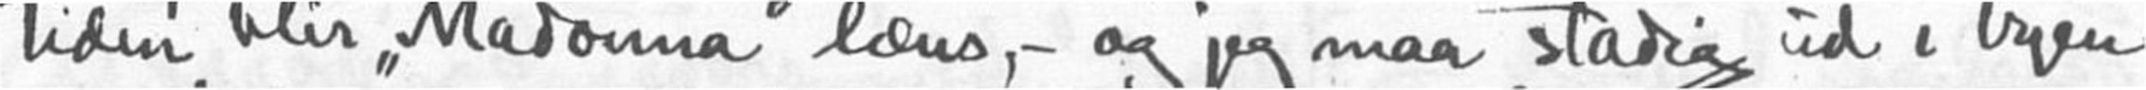tiden blir "Madonna" læns,- og jeg maa stadig ud i byen
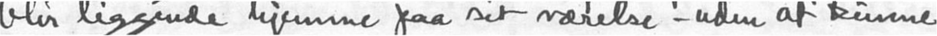blir liggende hjemme paa sit værelse - uden at kunne
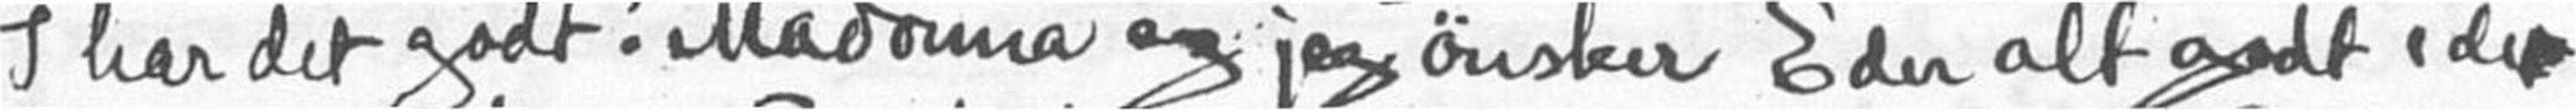I har det godt! Madonna og jeg ønsker Eder alt godt i de
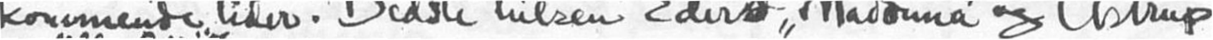kommende tider. Beste hilsen Eders "Madonna" og Astrup
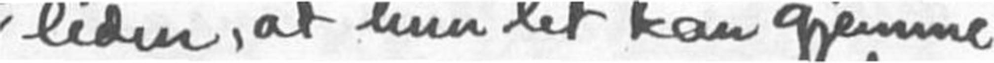liden, at hun let kan gjemme
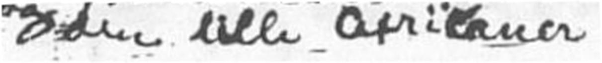og den lille Afrikaner.
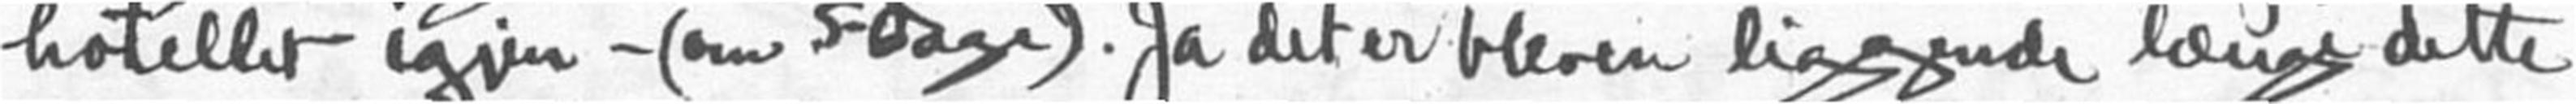hotellet igjen - (om 5-6 dage). Ja det er bleven liggende længe dette
